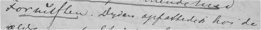Fornuften. Dyden opfattede hos de
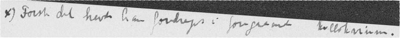*) Første del havde han foredraget i foregaaende kollokvium.
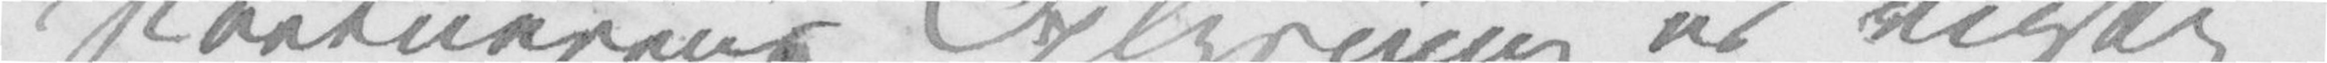Portnerens Oplysning er rigtig
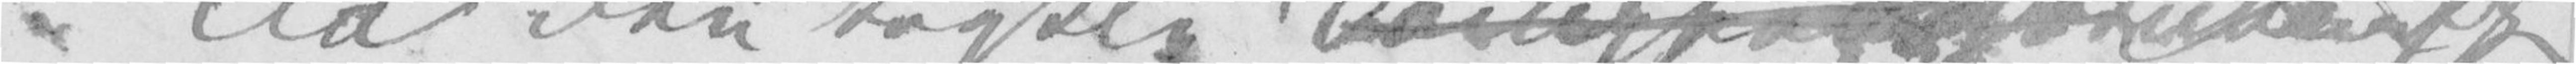da den trykte D<gap /></del>
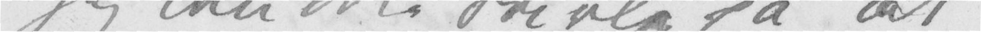Jeg kan ikke tvivle på at
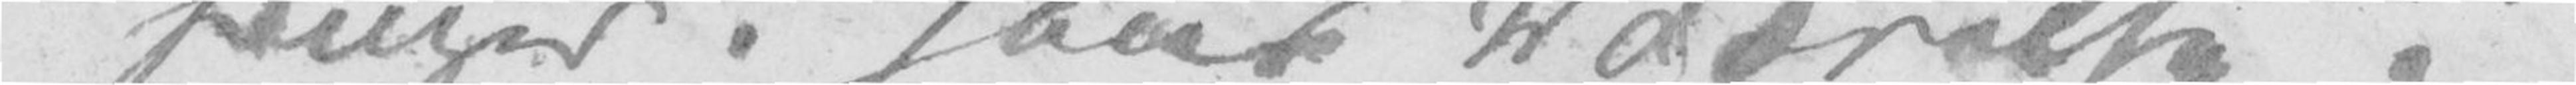henger i hans Værelse.
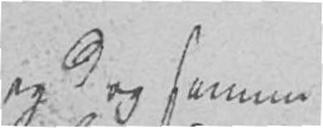og dog samme
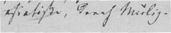asiatiske, deraf Mulig-
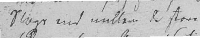Slags end mellem de store
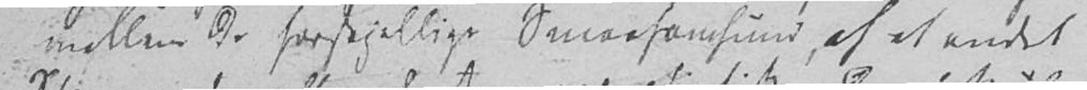mellem de forskjellige Smaasamfund, af et andet
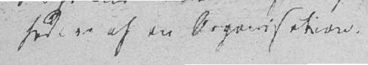heden af en Organisation.
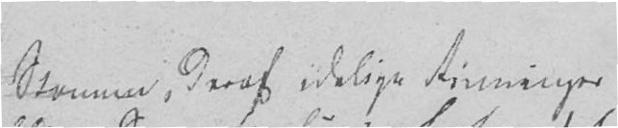Stammer, deraf idelige Rivninger
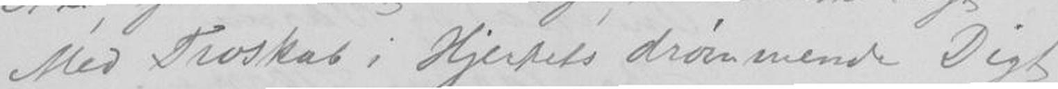Med Troskab i Hjertets drømmende Digt,
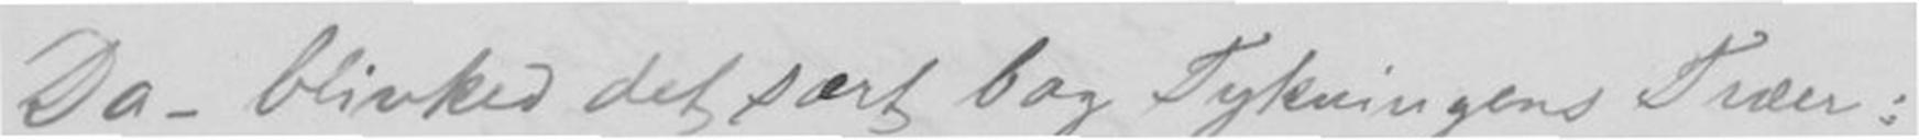Da - blinked det sært bag Tykningens Træer:
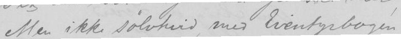Men ikke sølvhird, med Eventyrbogen,
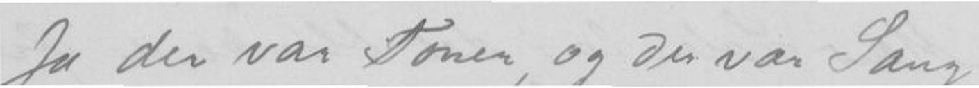Ja der var Toner, og der var Sang,
